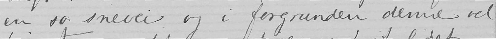en so snevei og i forgrunden denne vel
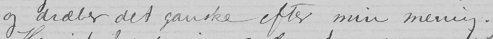og dræber det ganske efter min mening.
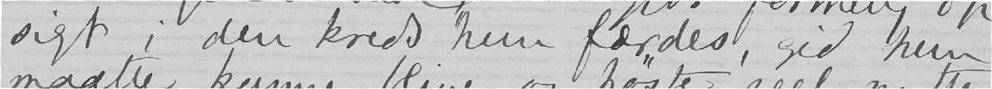sigt i den kreds hun færdes, gid hun
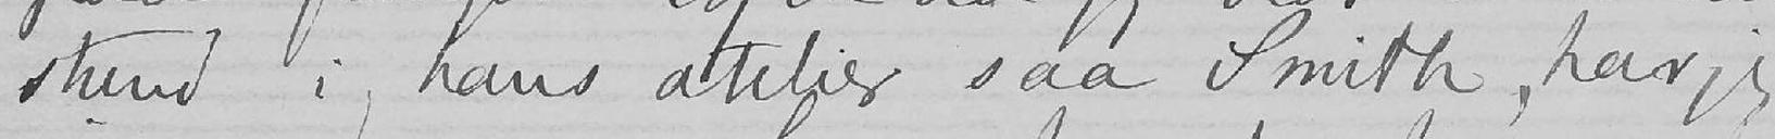stund i hans atelier saa Smith, har jeg
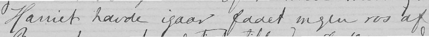Harriet havde igaar faaet megen ros af
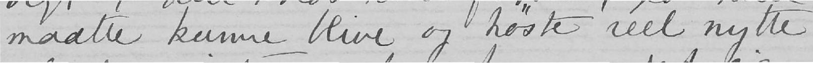maatte kunne blive og høste reel nytte
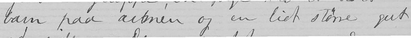barn paa armen og en lidt større gut
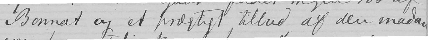Bonnat og et prægtigt tilbud af den madam
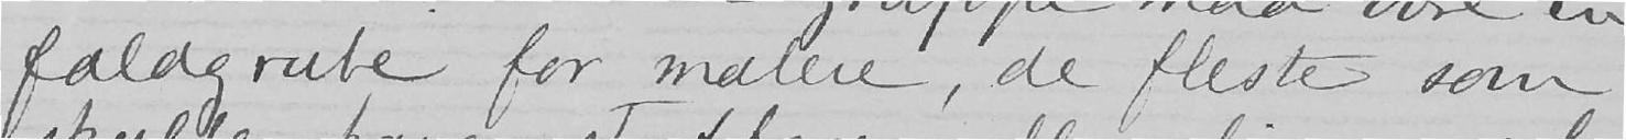faldgrube for malere, de fleste som
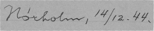Nørholm, 14/12. 44.
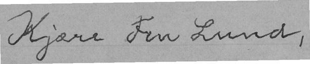Kjære Fru Lund,
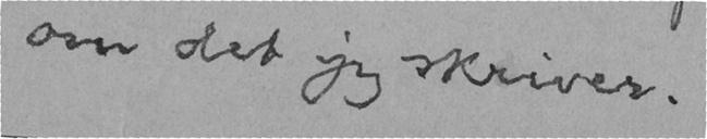om det jeg skriver.
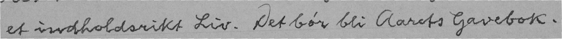et indholdsrikt Liv. Det bør bli Aarets Gavebok.
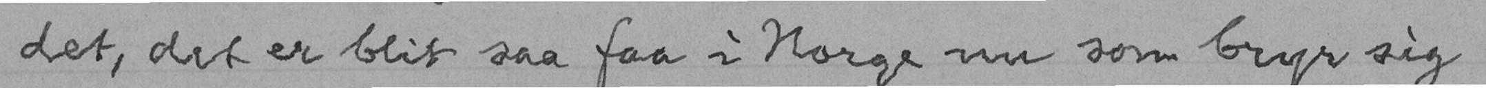det, det er blit saa faa i Norge nu som bryr sig
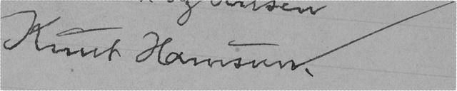Knut Hamsun.
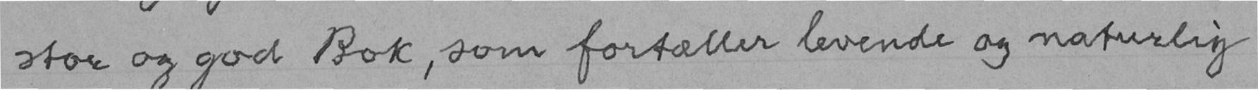stor og god Bok, som fortæller levende og naturlig
